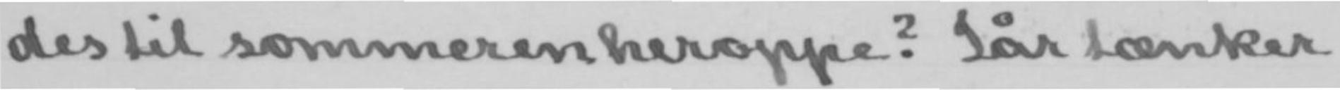des til sommeren heroppe? Iår tænker
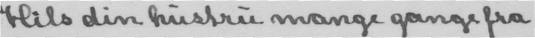Hils din hustru mange gange fra
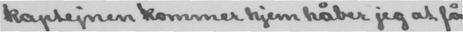kaptejnen kommer hjem håber jeg at få
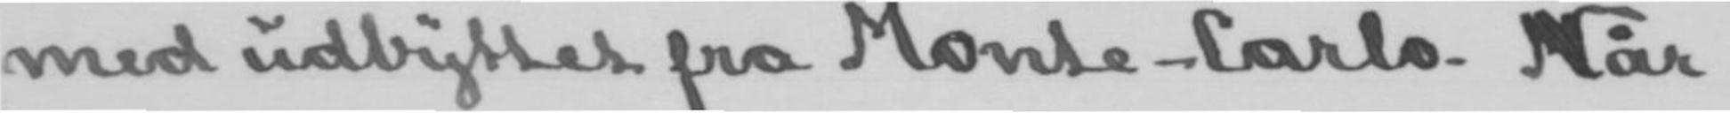med udbyttet fra Monte-Carlo. Når
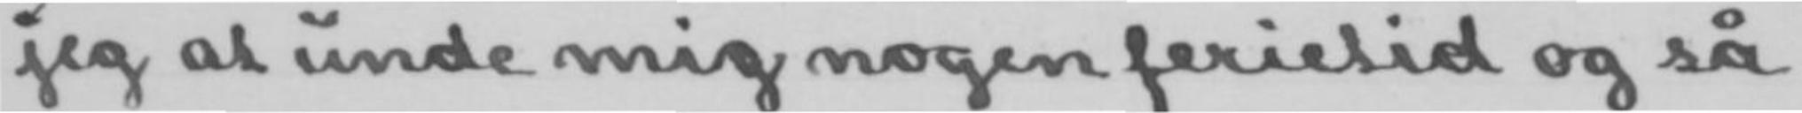jeg at unde mig nogen ferietid og så
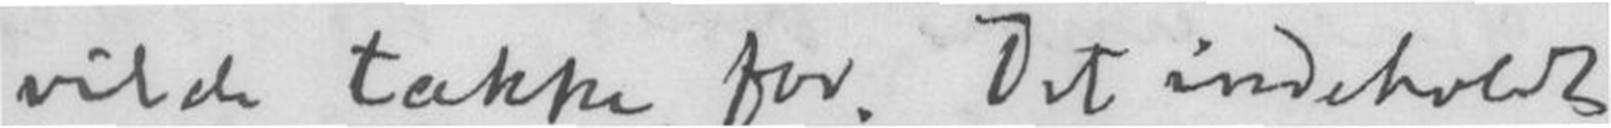vilde takke for. Det indeholdt
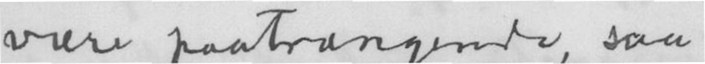være paatrængende, saa
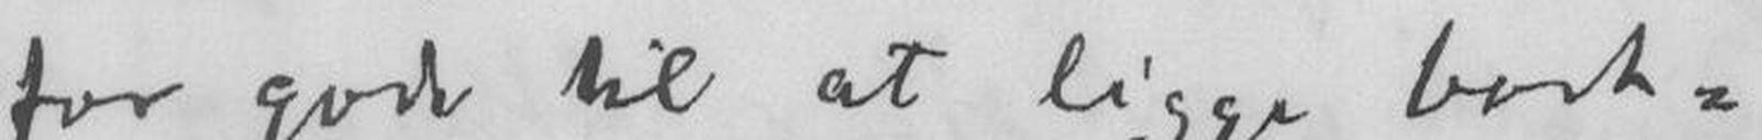for gode til at ligge bort-
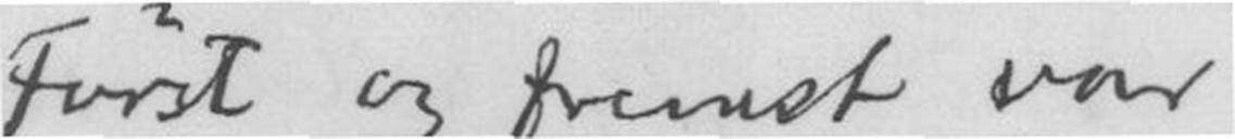Først og fremst var
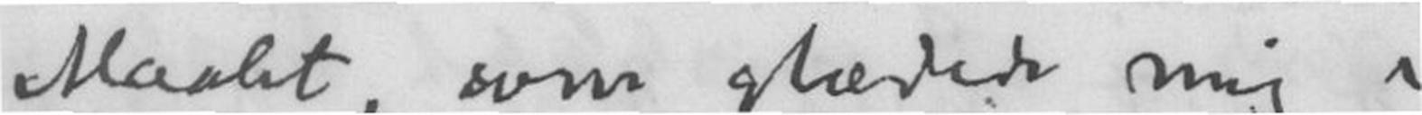Maalet, som glædede mig i
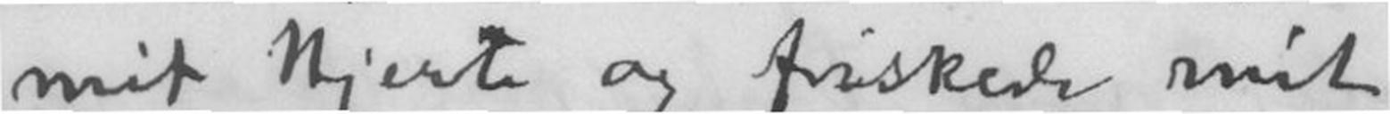mit Hjerte og friskede mit
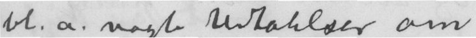bl. a. nogle Udtalelser om
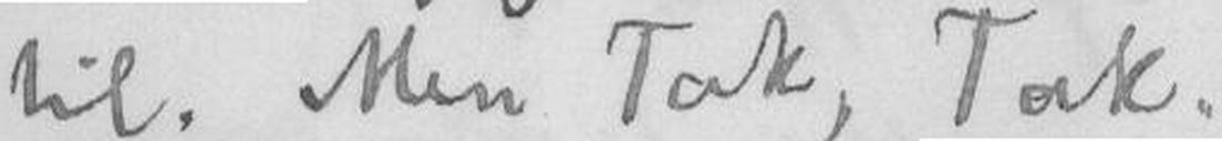til. Men Tak, Tak.
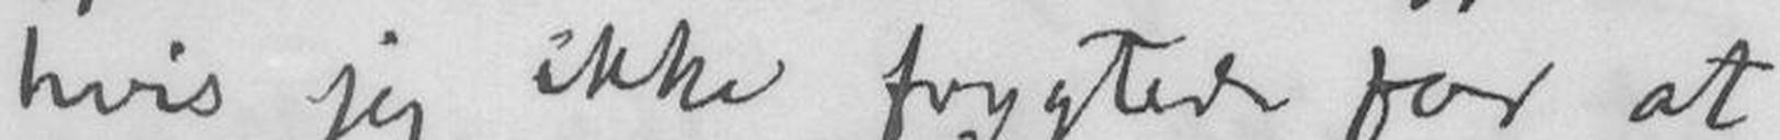hvis jeg ikke frygtede for at
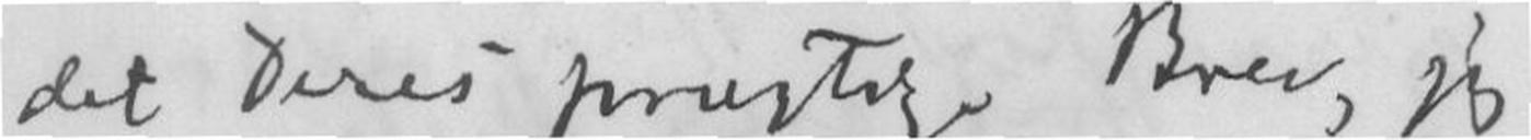det Deres prægtige Brev, jeg
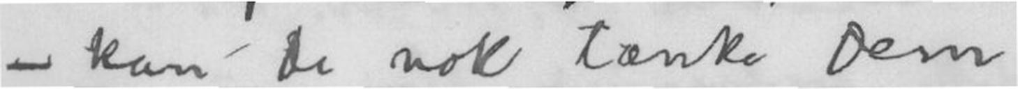- kan De nok tænke Dem
